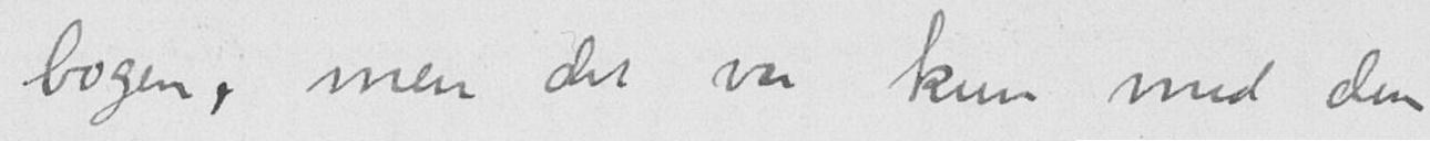bogen, men det var kun med den
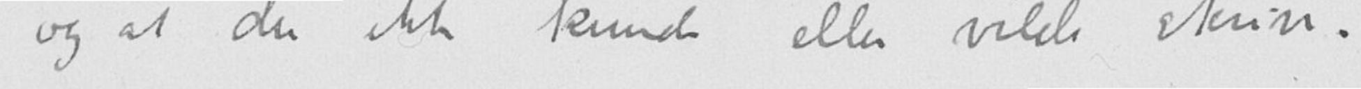og at du ikke kunde eller vilde skrive.
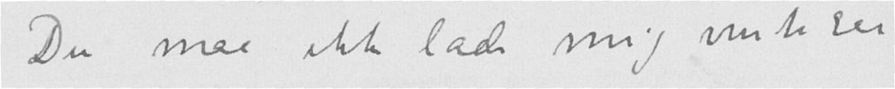Du maa ikke lade mig vente saa
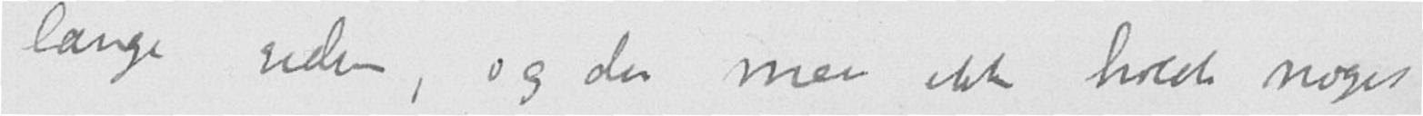længe siden, og du maa ikke holde noget
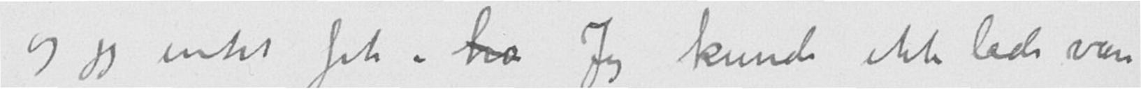og jeg intet fik. ha Jeg kunde ikke lade være
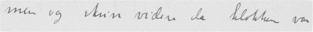men og skrive videre da klokken var
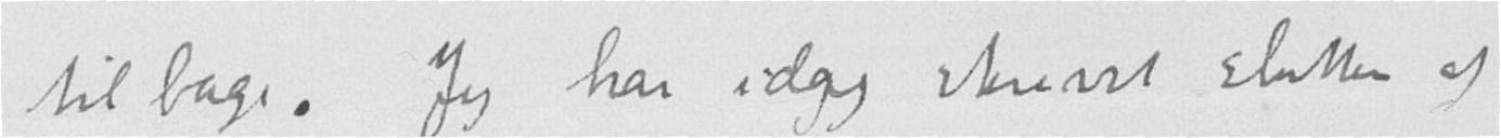tilbage. Jeg har idag skrevet slutten af
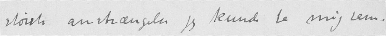største anstrængelse jeg kunde ta mig sam-
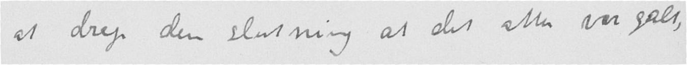at drage den slutning at det atter var galt,
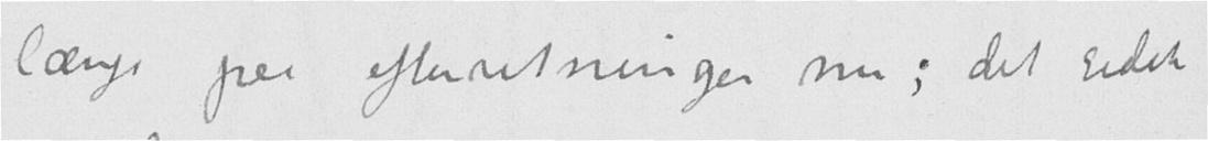længe paa efterretninger nu; det sidste
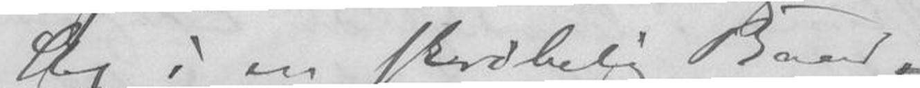Slig i en skrøbelig Baad,
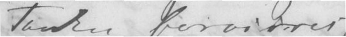Tanken forvirres,
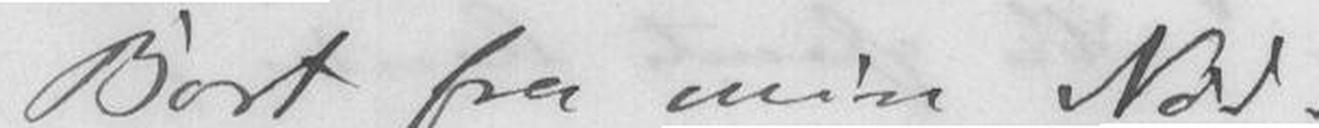Bort fra min Nød.
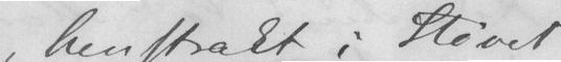, henstrakt i Støvet
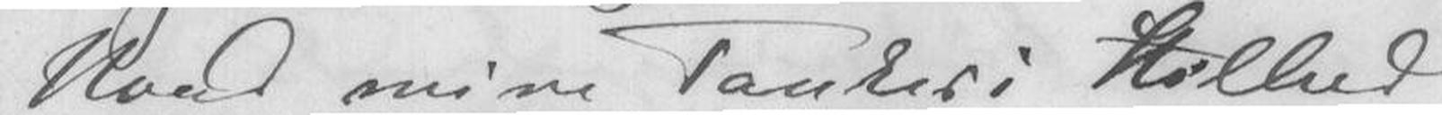Hvad mine Tanker i Stilhed
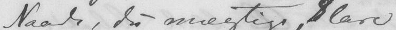Naade, du megtige klare
